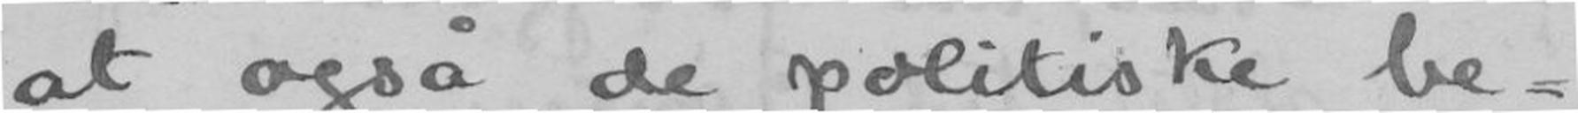at også de politiske be-
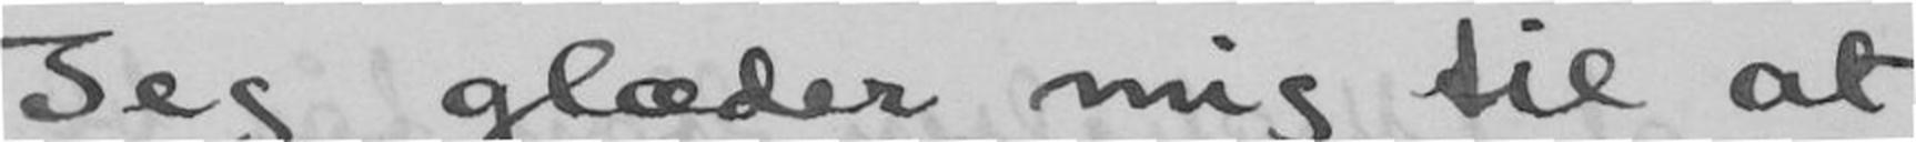Jeg glæder mig til at
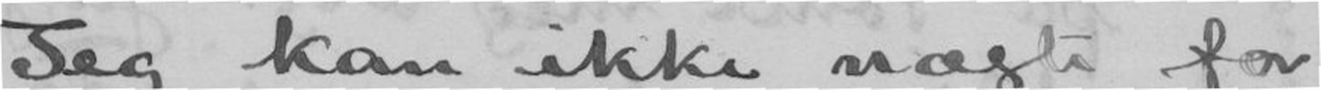Jeg kan ikke nægte for
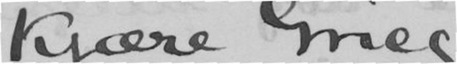Kjære Grieg!
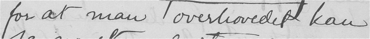for at man i overhovedet kan
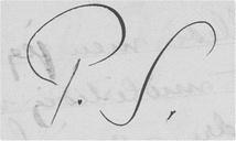P. S.
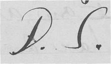D. S.
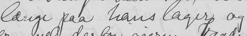længe paa hans lager og
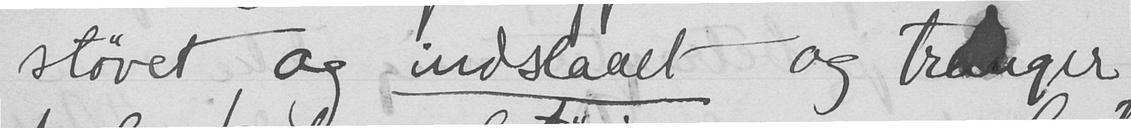støvet og indslaaet og trænger
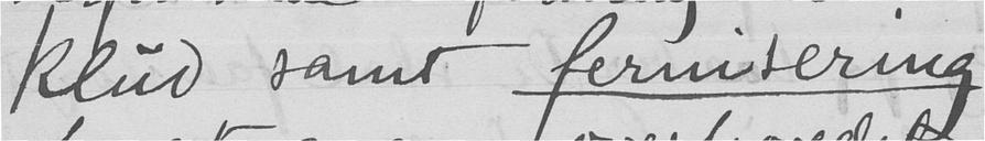klud samt fernisering
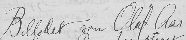Billedet som Olaf Aas
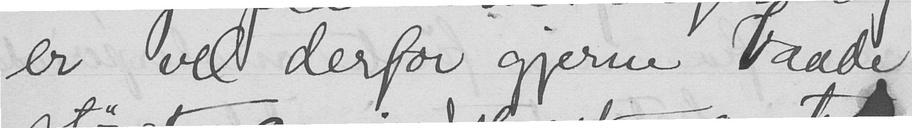er vel derfor gjerne baade
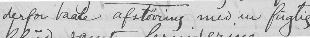derfor baade afstøving med en fugtig
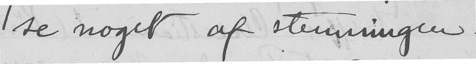se noget af stemningen.
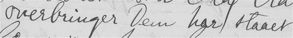overbringer Dem har staaet
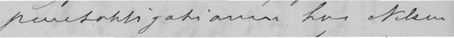Pantobligationen hos Nilsen
